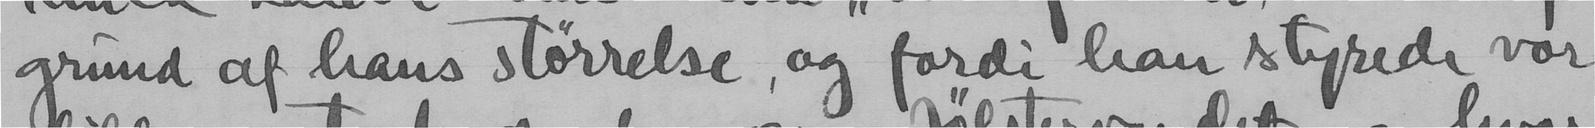grund af hans størrelse, og fordi han styrede vor
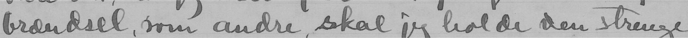brændsel, som andre, skal jeg holde den strenge
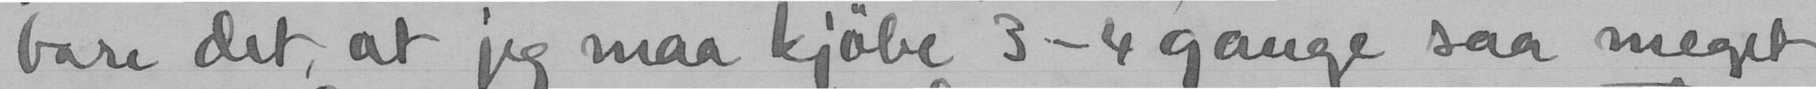bare det, at jeg maa kjøbe 3-4 gange saa meget
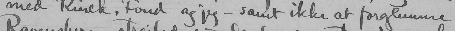med Kinck, Fond og jeg - samt ikke at forglemme
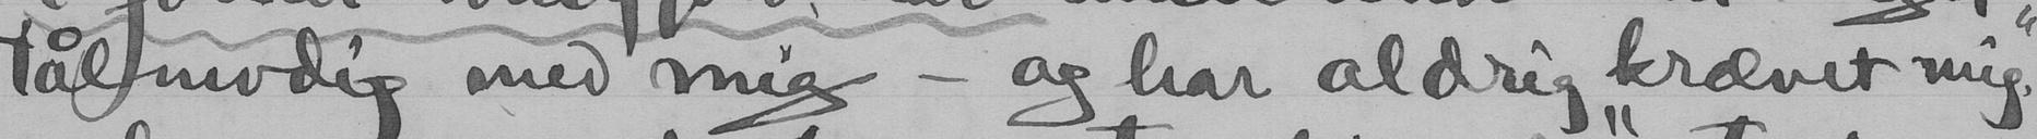tålmodig med mig - og har aldrig "krævet mig".
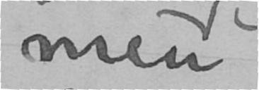men
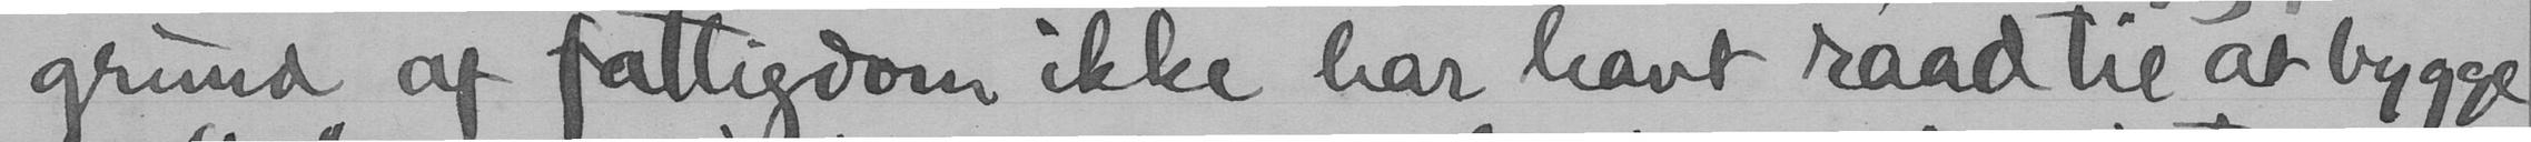grund af fattigdom ikke har havt raad til at bygge
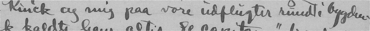Kinck og mig paa vore udflugter rundt i bygden.
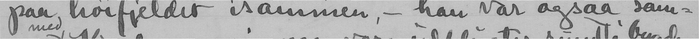paa høifjeldet isammen, - han var ogsaa sam-
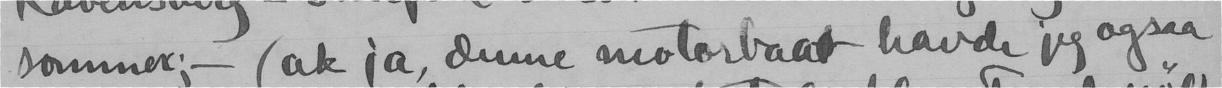sommer; - (ak ja, denne motorbaat havde jeg ogsaa
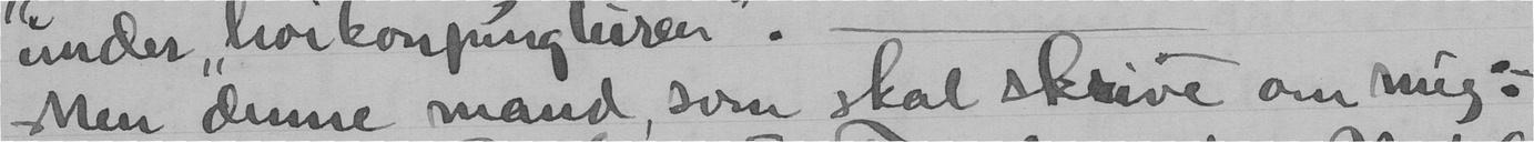Men denne mand, som skal skrive om mig:-
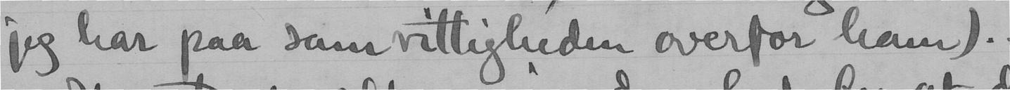jeg har paa samvittigheden overfor ham).
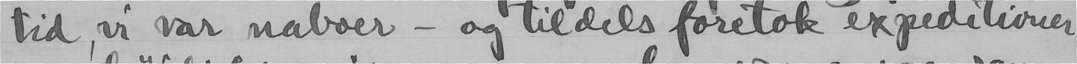tid, vi var naboer - og tildels foretok expeditioner
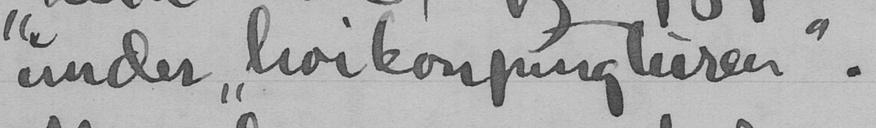under "hvikonpingturen".
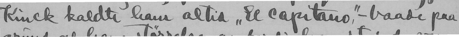Kinck kaldte hans altid "El Capitano" - baade paa
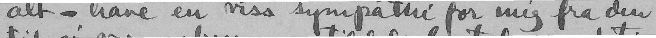alt - have en viss sympathi for mig fra den
